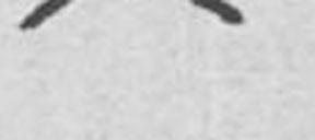x
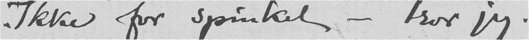Ikke for spinkel — tror jeg.
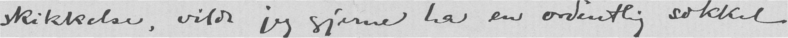skikkelse, vilde jeg gjerne ha en ordentlig sokkel
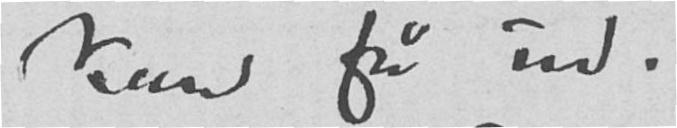kan få ud.
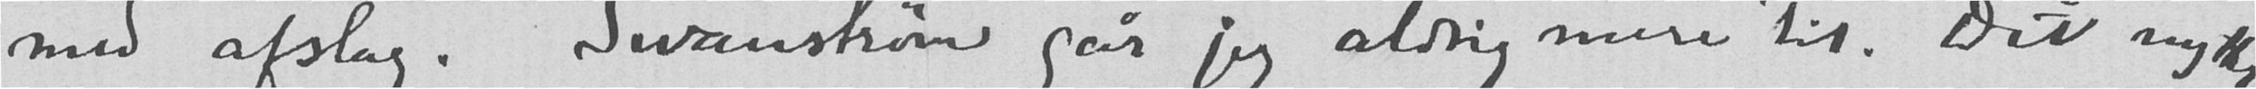med afslag. Swanstrøm går jeg aldrig mere til. Det nytter
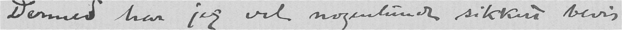Dermed har jeg vel nogenlunde sikkert bevis
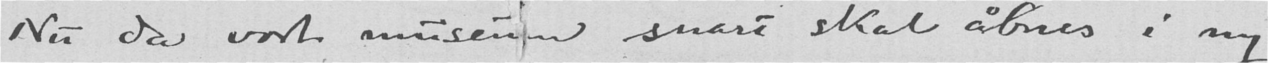Nu da vort museum snart skal åbnes i ny
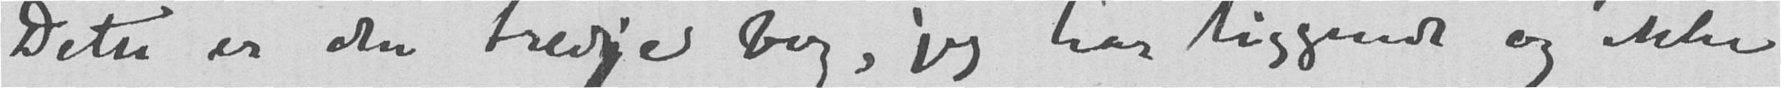Dette er den tredje bog, jeg har liggende og ikke
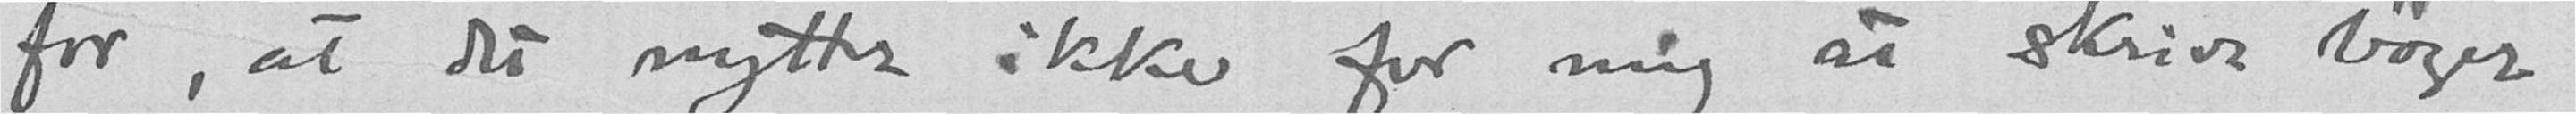for, at det nytter ikke for mig at skrive bøger.
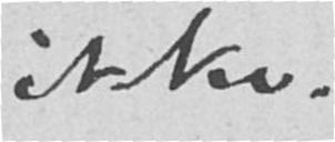ikke.
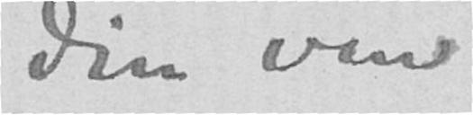Din ven
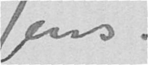Jens
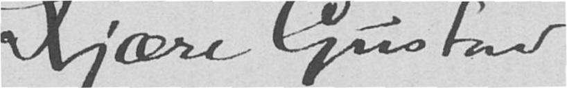Kjære Gustav
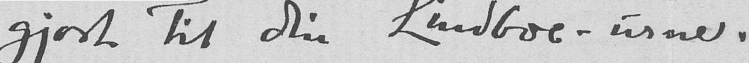gjort til din Lindboe-urne.
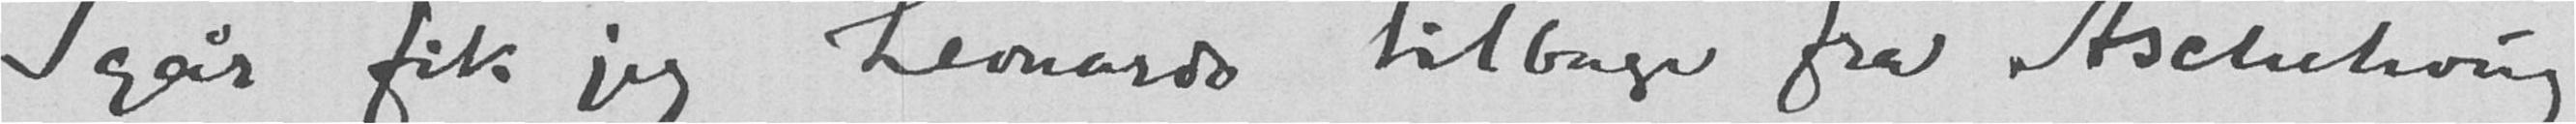Igår fik jeg "Leonardo" tilbage fra Aschehoug
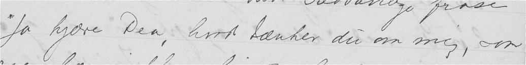: "Ja kjære Dea, hvad tænker du om mig, om
